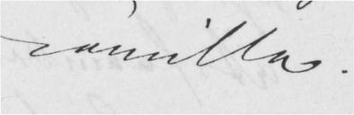Camilla.
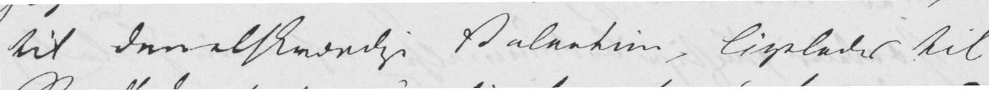til den elskværdige Valentine, ligeledes til
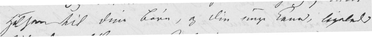Hilsen til Dine Børn, og Din unge Kone, ligeledes
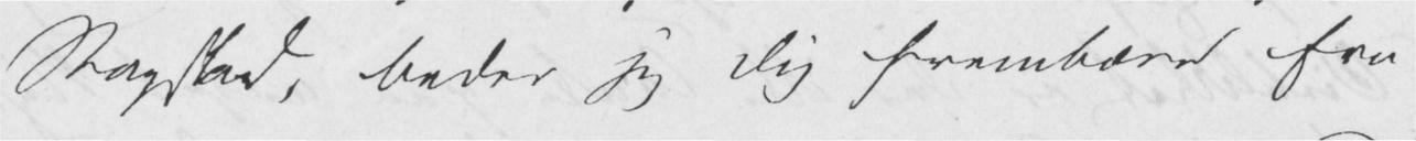Rogstad, beder jeg Dig frembære fra
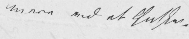mere end et Ønske.
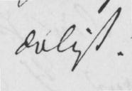ærligt!
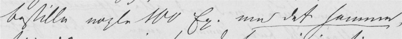bestille nogle 100 Ex. med det samme,
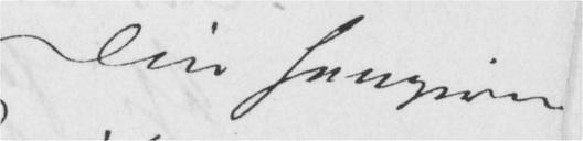Din hengivne
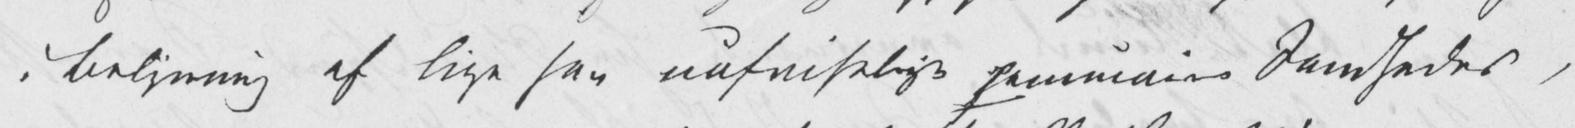i Belysning af lige saa uafviselige pecuniaire Sandheder,
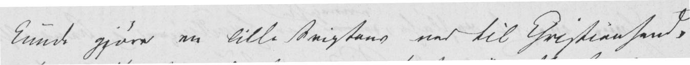kunde gjøre en lille Sviptour ned til Christiansand,
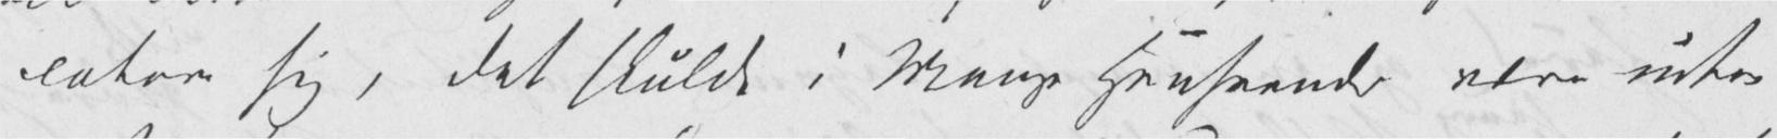datere sig, det skulde i Mange Henseender være inte-
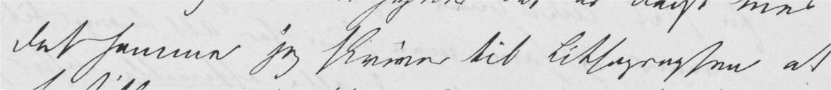det samme jeg skriver til Lithographen at
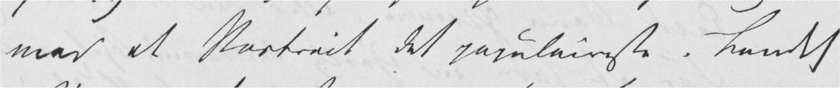med et Portrait det populaireste i Landet
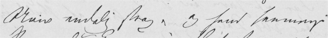Skriv endelig strax, og send saamange
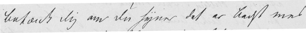Betænk Dig om Du synes det er bedst med
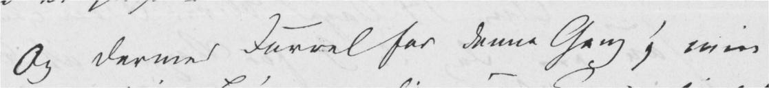Og dermed Farvel for denne Gang; min
